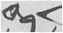og
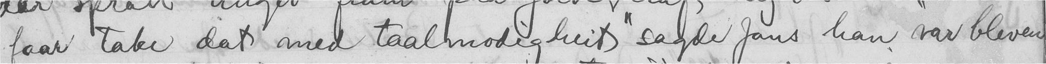faar take dat med taalmodigheit" sagde Jans han var bleven
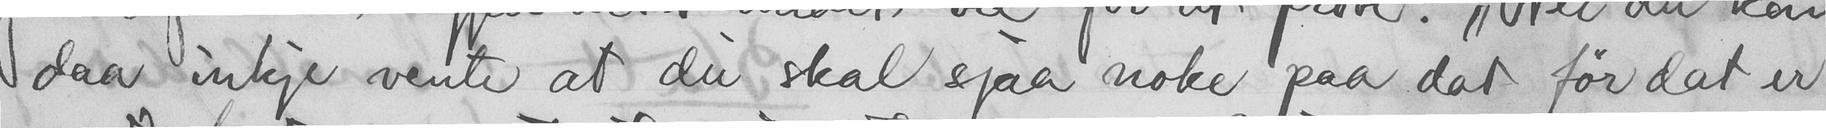daa inkje vente at du skal sjaa noke paa dat før det er
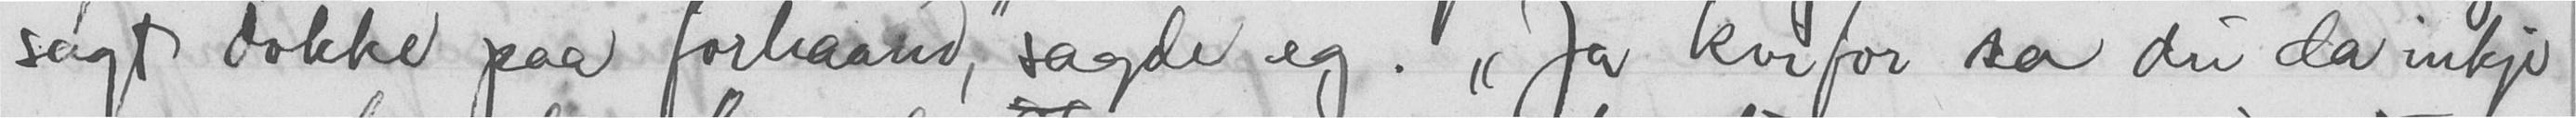sagt dokke paa forhaand", sagde eg. "Ja kvifor sa du da inkje
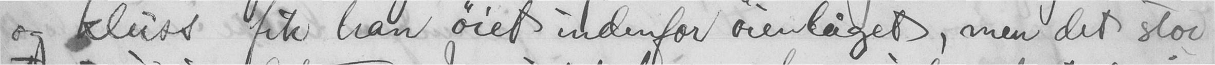og kluss fik han øiet indenfor øienluget, men det stoe
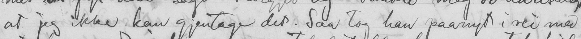at jeg ikke kan gjentage det. Saa tog han paanyt i vei med
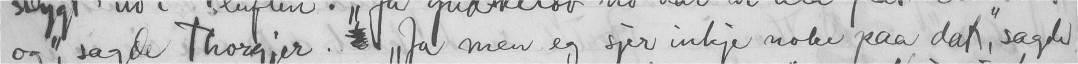og", sagde Thorgjer.  "Ja men eg sjer inkje noke paa dat," sagde
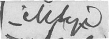ikkje
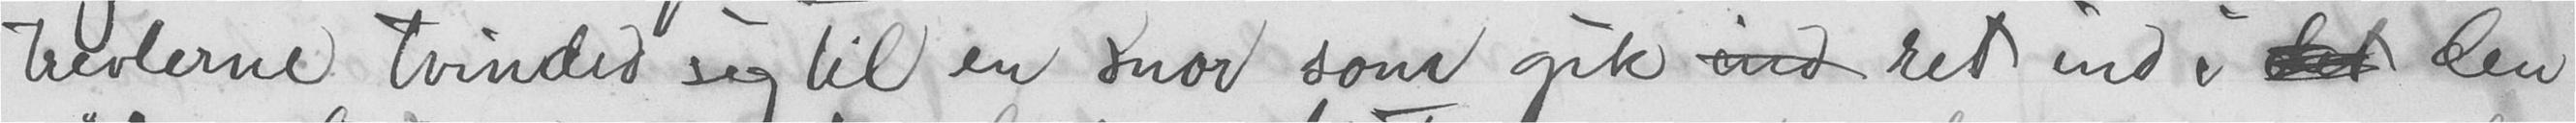trevlerne tvinded sig til en snor som gik  ret ind i  den
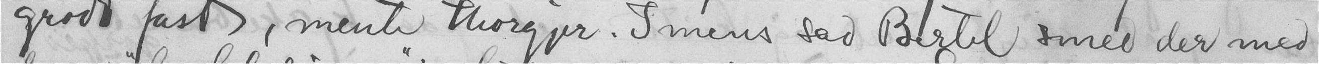grodd fast , mente Thorgjer. I mens sad Bertel smed der med
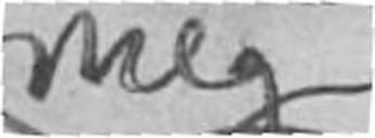meg
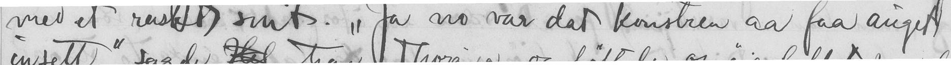med et raskt snit. "Ja no var dat konstren aa faa auget
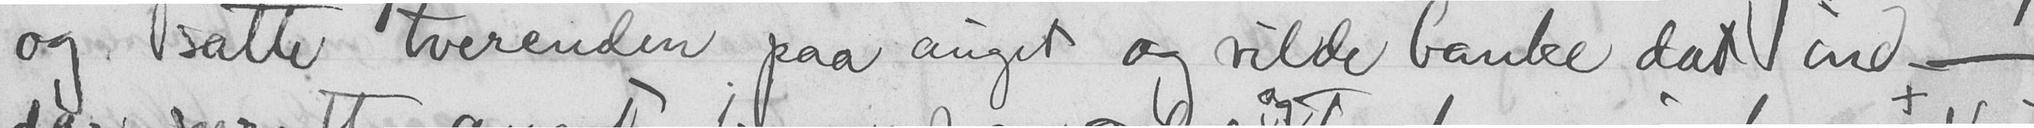og satte tverenden paa auget og vilde banke dat ind -
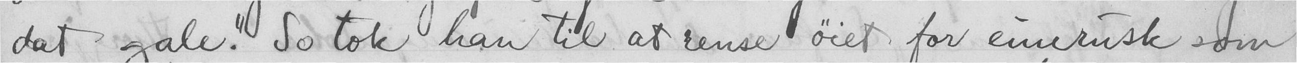dat gale." So tok han til at rense øiet for einerusk som
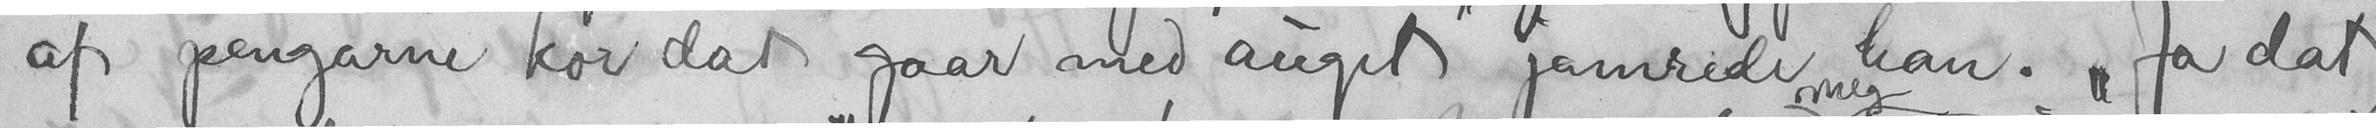af pengarne kor dat gaar med auget" jamrede han. "Ja dat
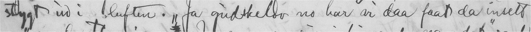stygt ud i luften. "Ja gudskelov no har vi daa faat da insett
